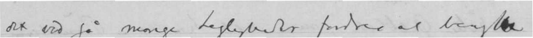det ved så mangen Lejligheder fodres at benytte
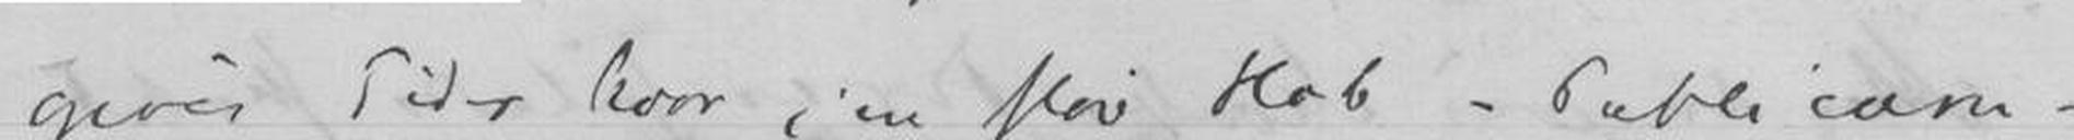gives Tider hvor i en sløv Hob - Publicum -
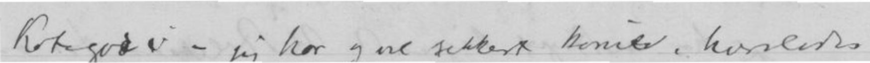Kategori - jeg har og vil sikkert kome, hvorledes
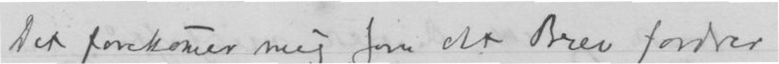Det forekomer mig som det Brev fordrer
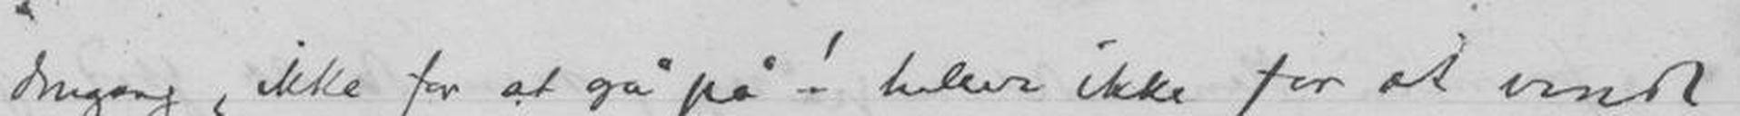dengang, ikke for at gå på! heller ikke for at vinde
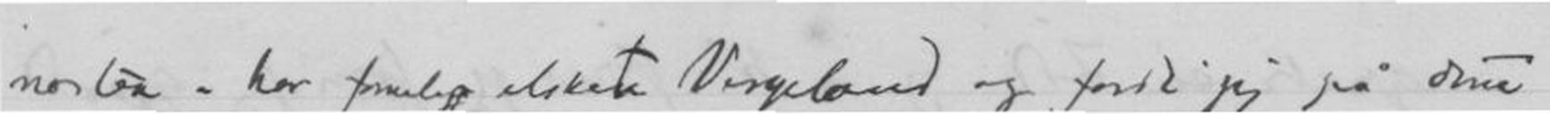næsten - har formelig elskede Vergeland og fordi jeg på denne
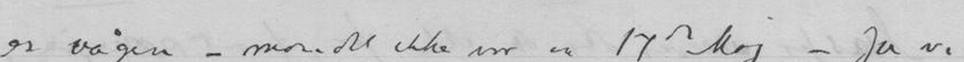er vågen - mon det ikke var en 17de Maj - Ja vi
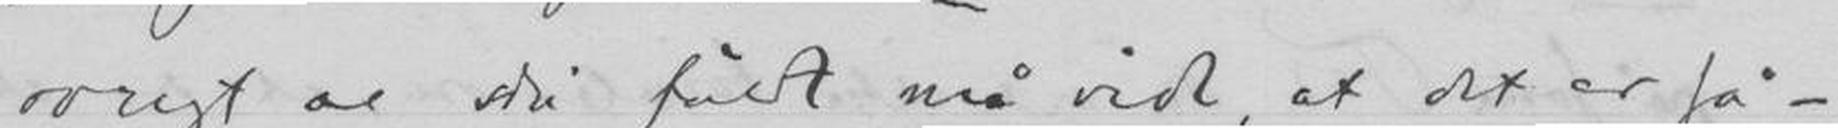øvrigt at du fuldt må vide, at det er så-
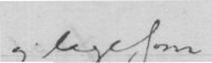og ligesom
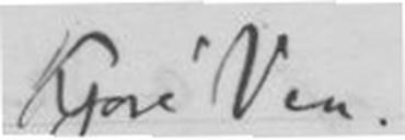Kjære Ven.
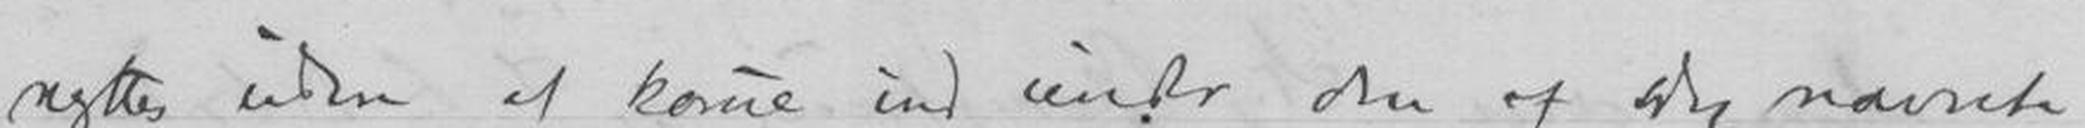nyttes uden at kome ind under den af Dig nevnte
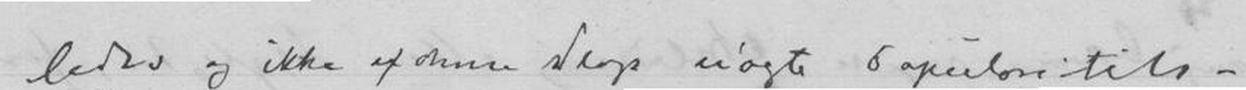ledes og ikke af denne slags uøgte sopuloritits-
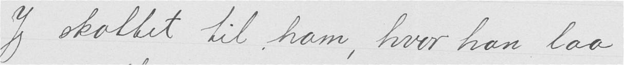Jeg skottet til ham, hvor han laa
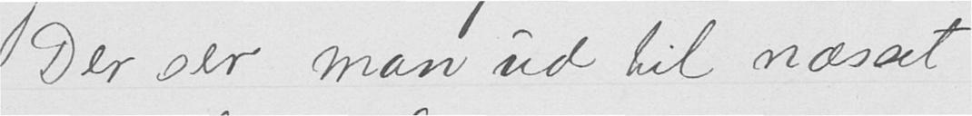Der ser man ud til næsset
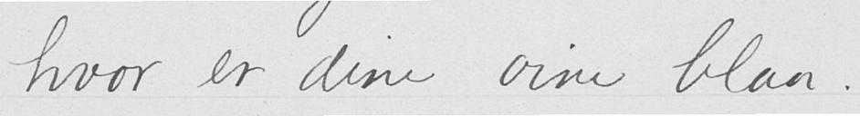hvor er dine øine blaa!
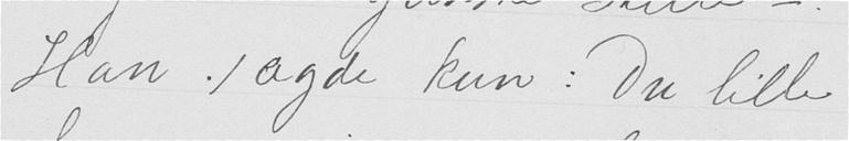Han sagde kun: Du lille
